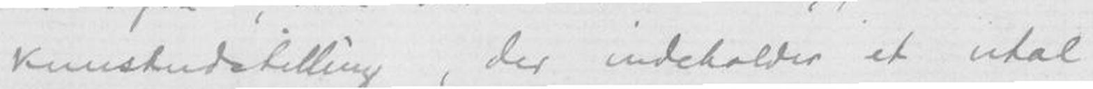kunstudstilling, der indeholder et utal
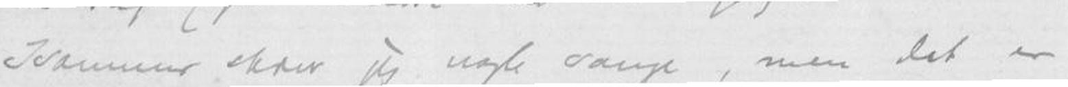Isommer skrev jeg nogle sange, men det er
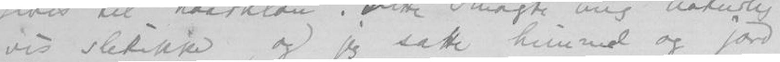vis slet ikke, og jeg satte himmel og jord
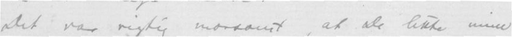Det var rigtig morsomt, at De likte mine
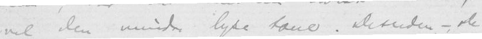vil den mindre lyse tone. Desuden - De
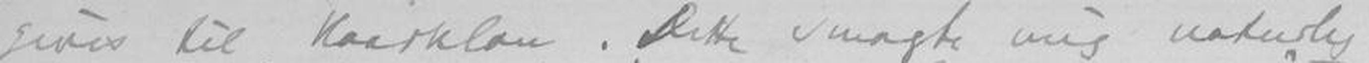gives til Haarklou. Dette smagte mig naturlig-
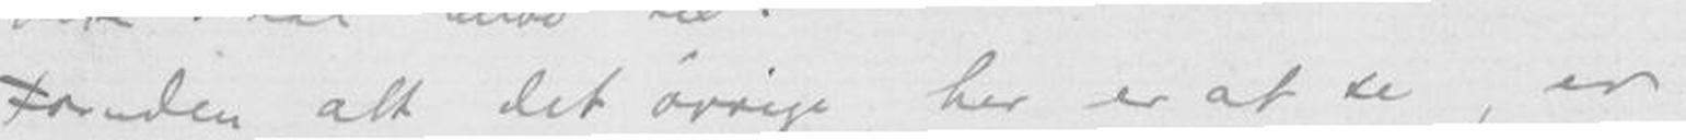Foruden alt det øvrige her er at se, er
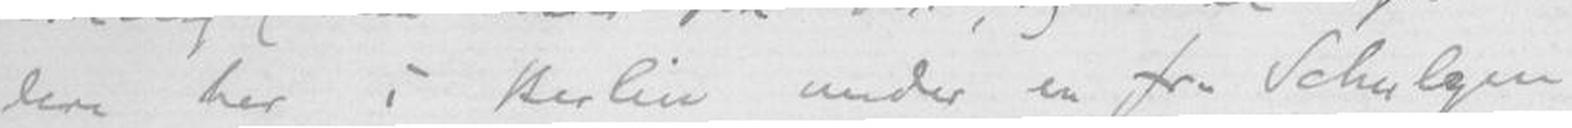dere her i Berlin under en fr. Schulgen
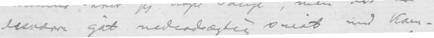desværre gåt nederdrægtig småt med kom-
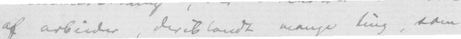af arbeider, deriblandt mange ting, som
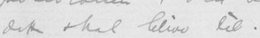dette skal blive til.
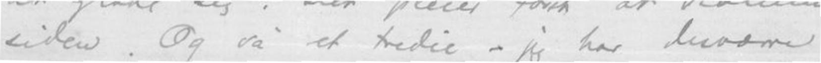siden. Og så et tredie - jeg har desværre
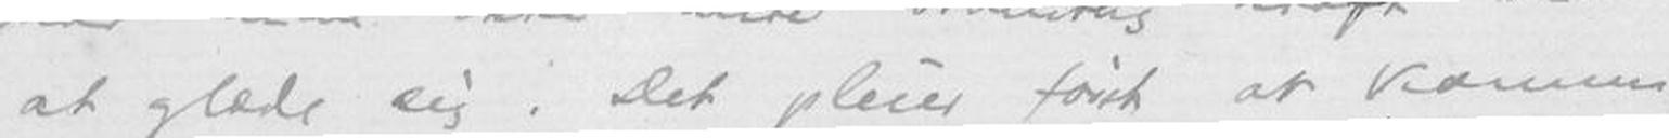at glæde sig. Det pleier først at komme
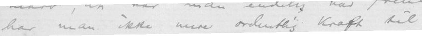har men ikke mere ordentlig kraft til
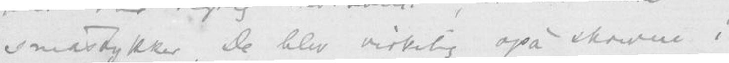småstykker. De blev virkelig også skrevne i
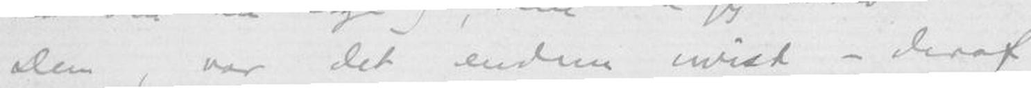Dem, var det endnu uvist - deraf
